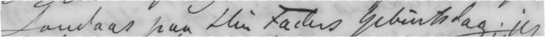Landaas paa Din Faders Geburtsdag; jeg
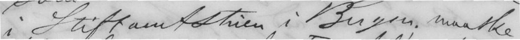i Stiftamtstuen i Bergen; maaske
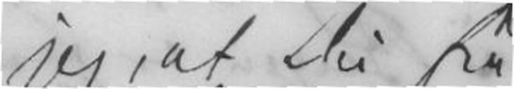jeg, at Du fra
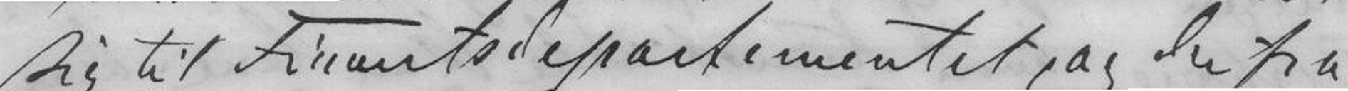sig til Finantsdepartementet, og derfra
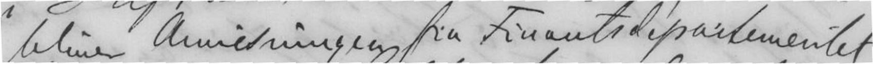bliver Anvisningen fra Finantsdepartementet
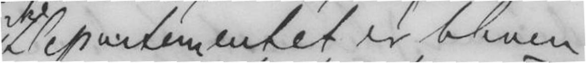Departemantet er bleven
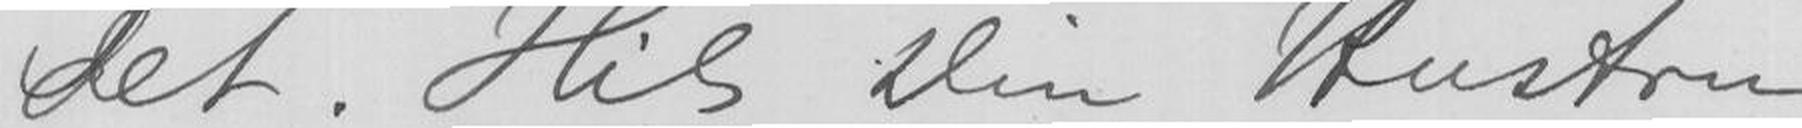det. Hils Din Hustru
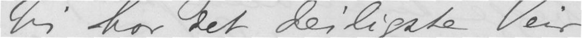Vi har det deiligdte Veir
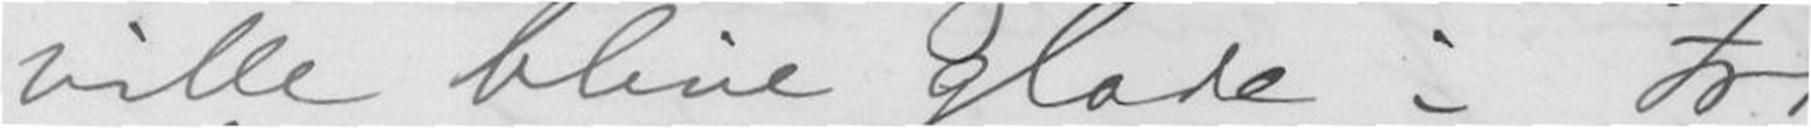ville blive glade i Frk
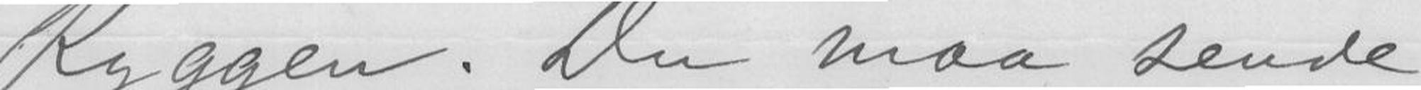Ryggen. Du maa sende
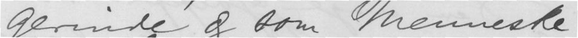gerinde og som Menneske.
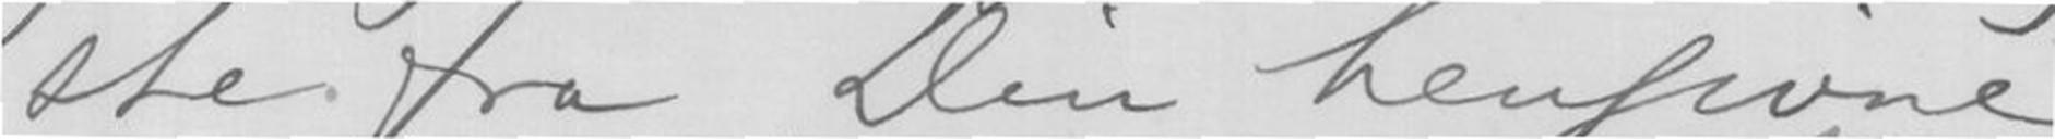ste fra Din hengivne
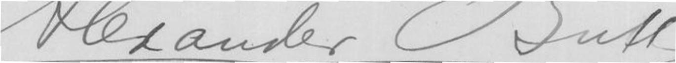Alexander Bull
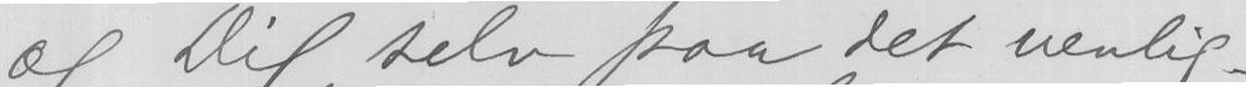og Dig selv paa det venlig-
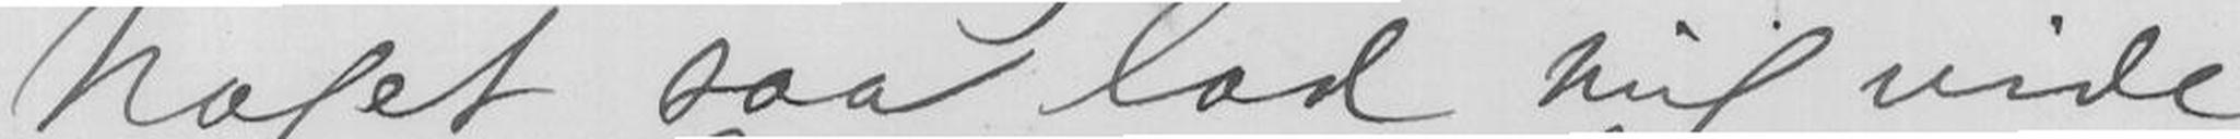Noget saa lad mig vide
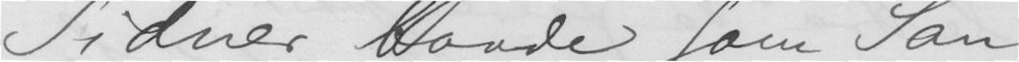Sidner, baade som San-
